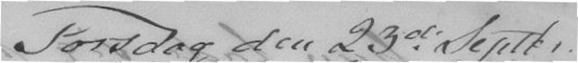Torsdag den 23de Septbr.
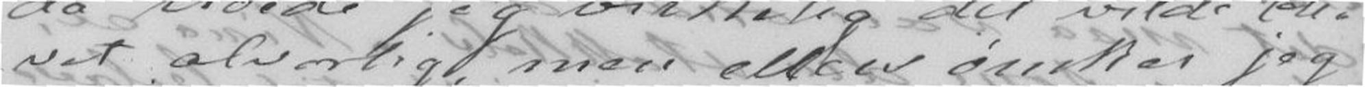vet alvorlig, men ellers ønsker jeg
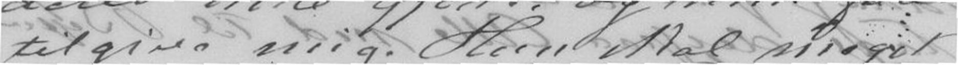tilgive mig. Hun skal meget
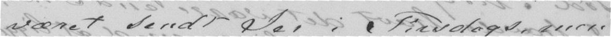været sendt Jer i Tirsdag, men
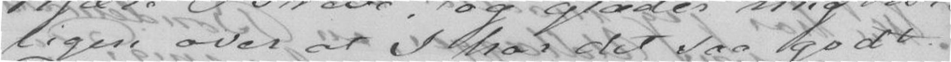ligen over at I har det saa godt.
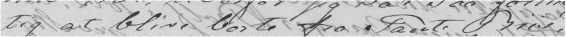tig at blive borte fra Tante Riis,
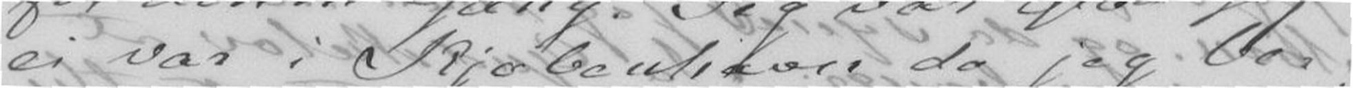ei var i Kjøbenhavn da jeg be-
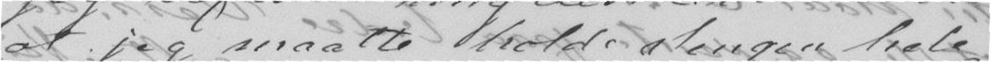at jeg maatte holde Sengen hele
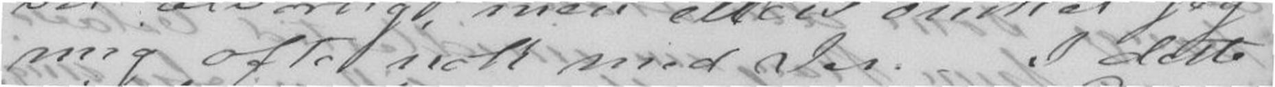mig ofte nok med Jer. I dette
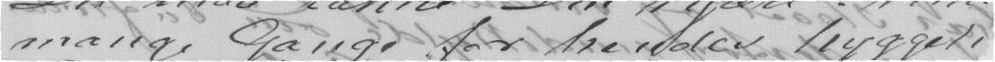mange Gange for hendes hyggeli
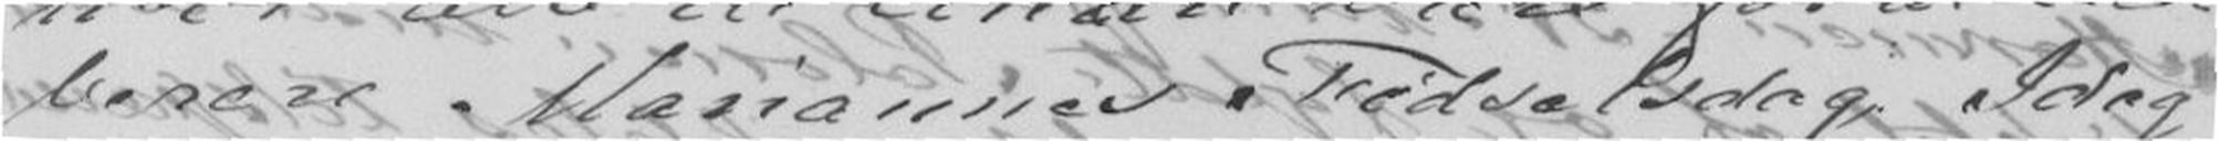berere Mariannes Fødselsdag. Idag
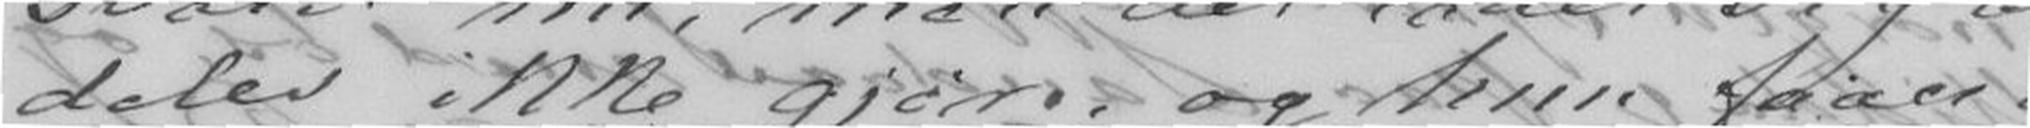deles ikke gjøre, og hun faaer
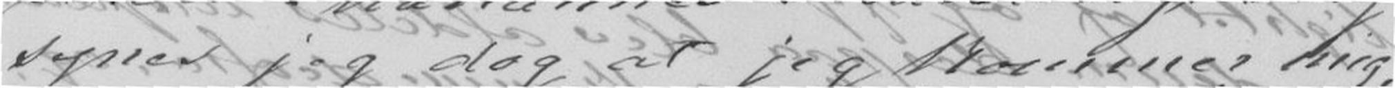synes jeg dog at jeg kommer mig,
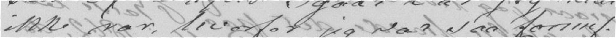ikke rar, hvorfor jeg var saa fornuf-
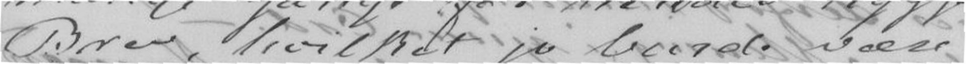Brev, hvilket jo burde være
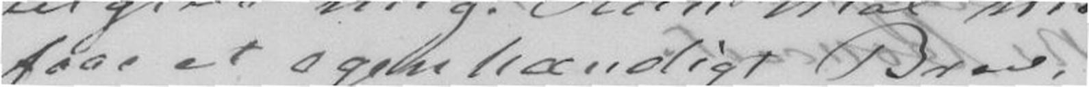faae et egenhændigt Brev, men
